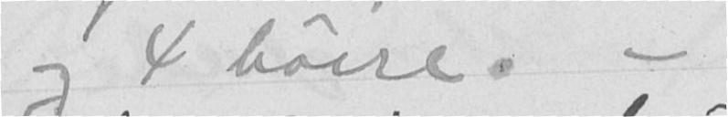og 4 høire. -
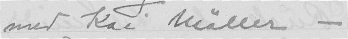med Kai Møller -
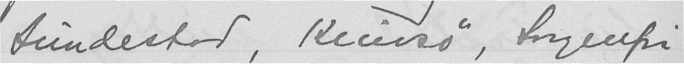Lundestad, Knivsø, Sorgenfri
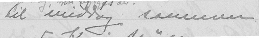til middag sammen
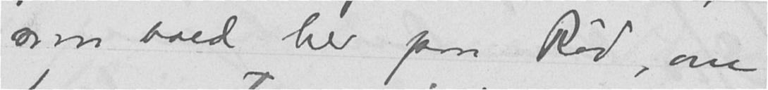som boed her paa Rød, om
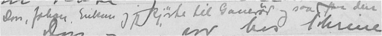Don, Johan, Eriksen og jeg kjørte til Ganerød og saa paa den
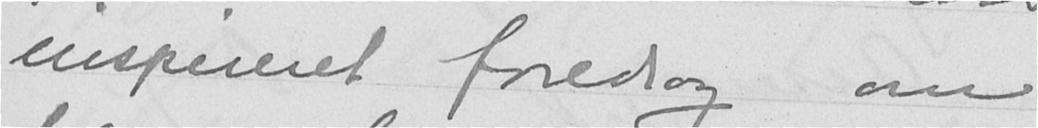inspireret foredrag om
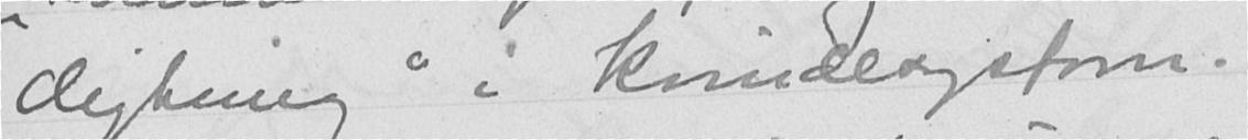digtning" i kvindesagsforn.
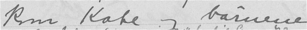kom Kate og børnene
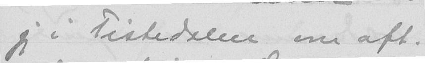jeg i Tistedalen om aft.
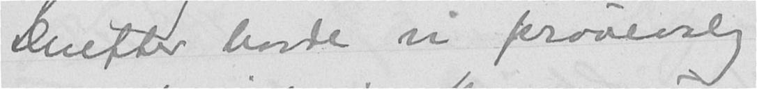Derefter havde vi prøvevalg
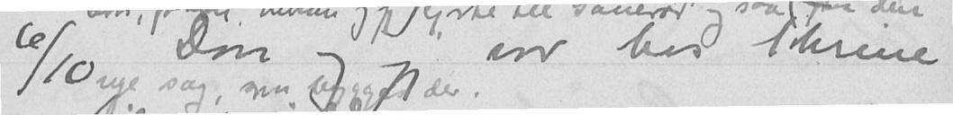6/10 Don og jeg var hos Thrine
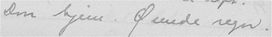Don hjem. Øsende regn.
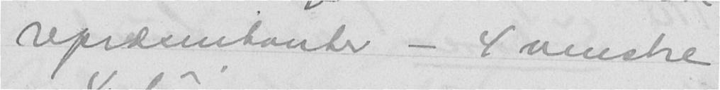repræsentanter - 4 venstre
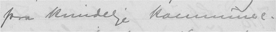paa kvindelige kommune-
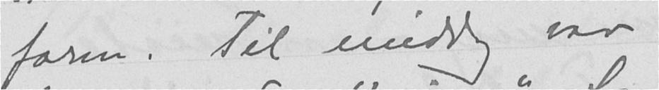form. Til middag var
